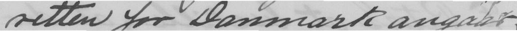retten for Danmark angaar,
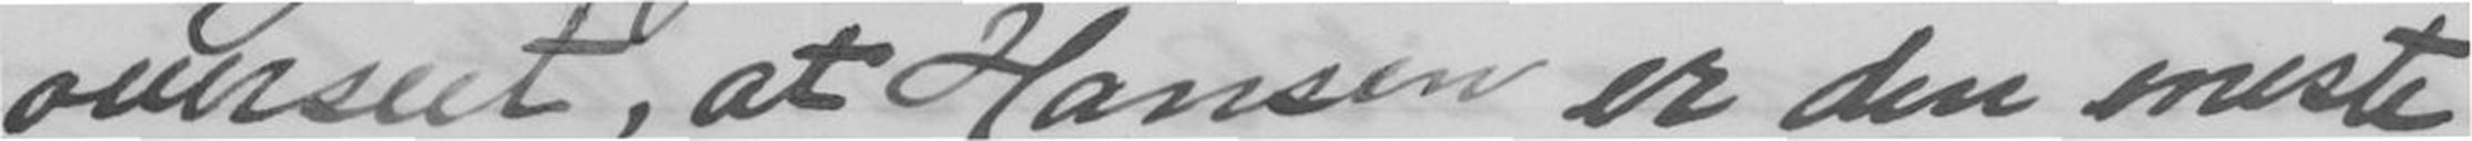overseet, at Hansen er den eneste
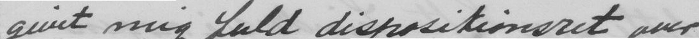givet mig fuld dispositionsret over
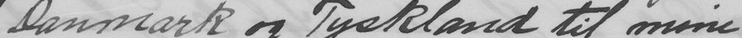Danmark og Tyskland til mine
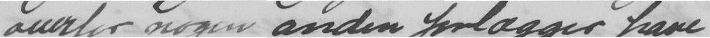overfor nogen anden forlægger have
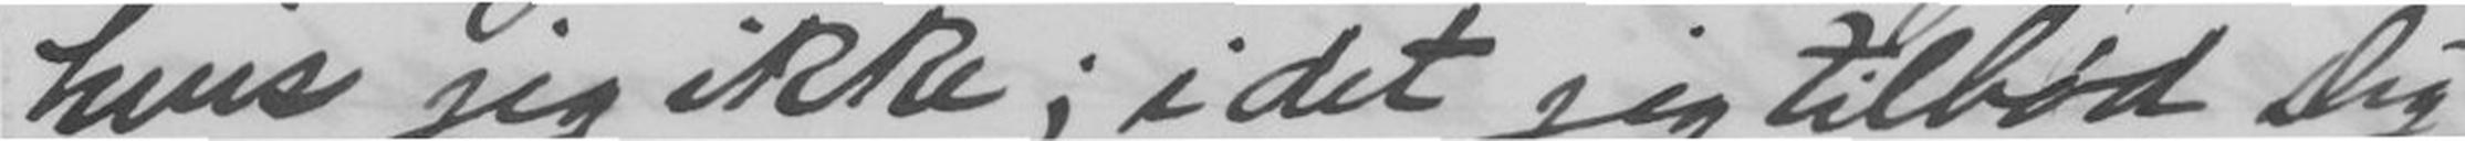hvis jeg ikke; i det jeg tilbød Dig
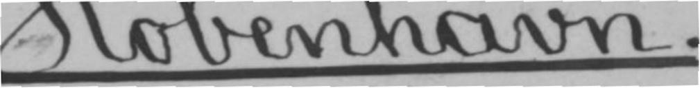København.
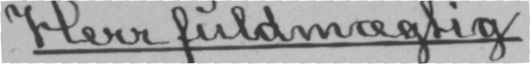Herr fuldmægtig
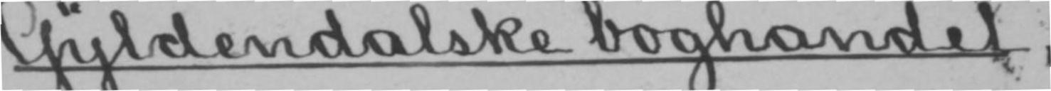Gyldendalske boghandel,
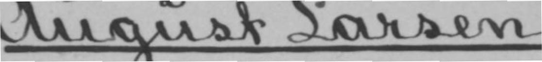August Larsen,
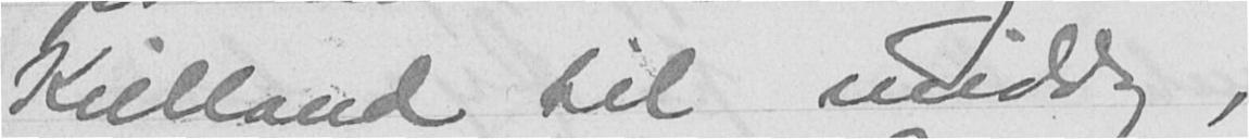Kielland til middag,
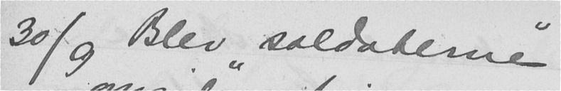30/9 Blev "soldaterne"
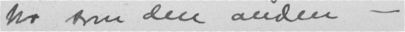tro som den anden -
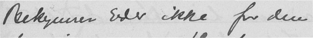Bekymrer Eder ikke for den
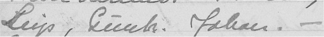Seips, Gunh. Johan. -
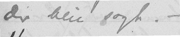der blir sagt. -
